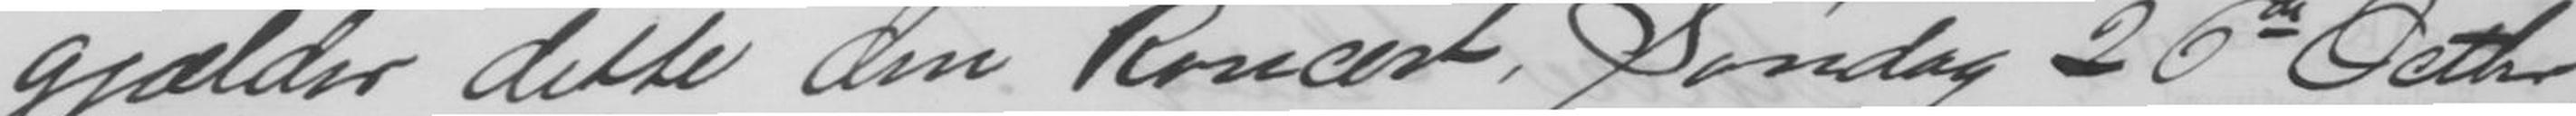gjælder dette din koncert, Søndag 26de Octbr.
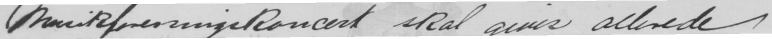Musikforeningskoncert skal gives allerede
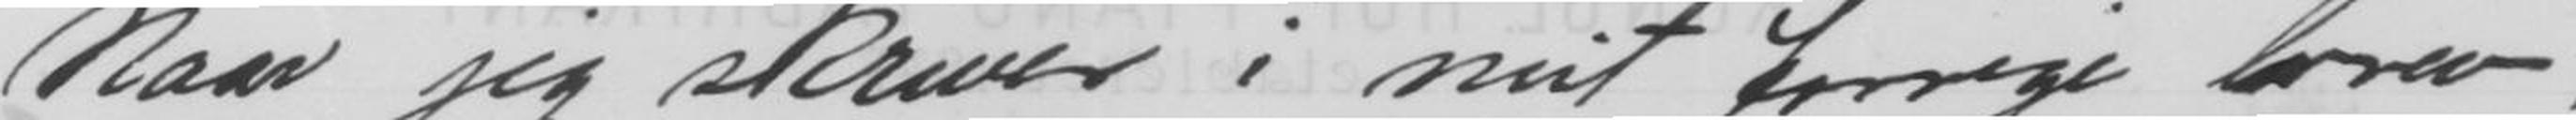Naar jeg skriver i mit forrige brev,
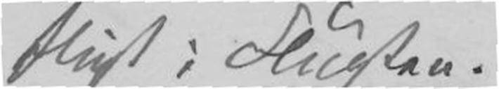sligt i Flugten.
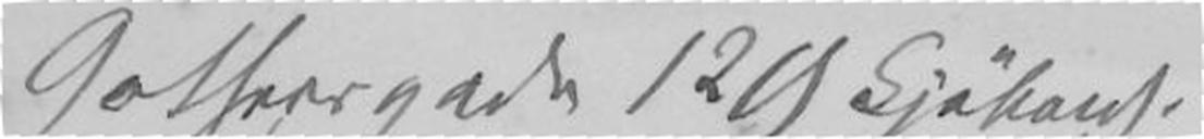Gotherrgade 12 G Kjøbenh
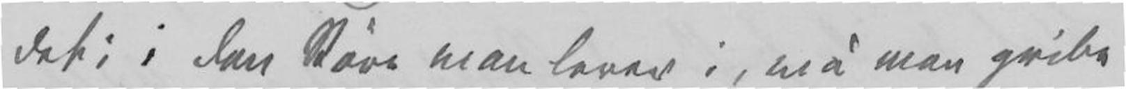det; i den Støve man lever i, må man gribe
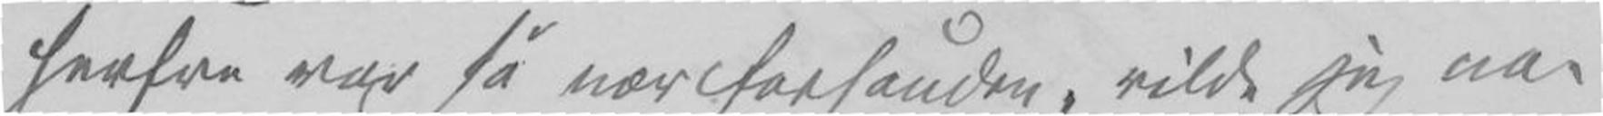herfra var så nær forhånden, vilde jeg na-
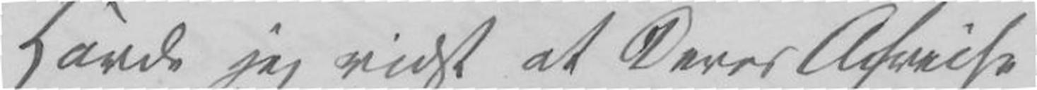Havde jeg vist at Deres Afreise
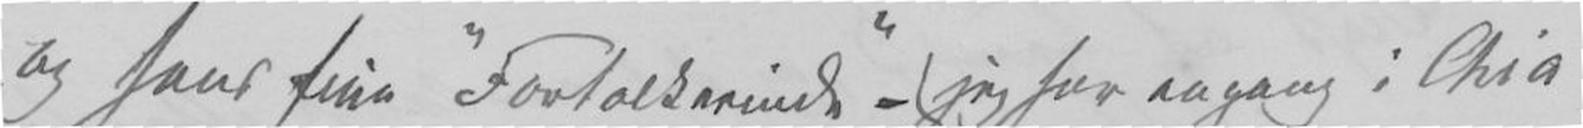og hans fine "Fortolkerinde" - (jeg har engang i Chia
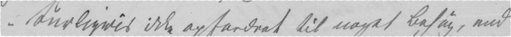-turligvis ikke opfordret til noget besøg, end
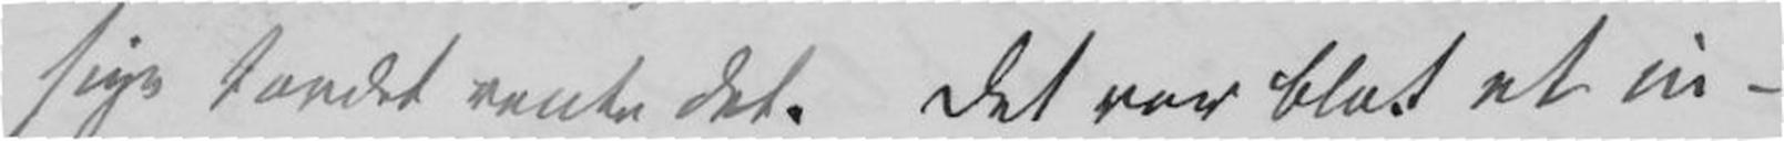sige turdet vente det. Det var blot et in-
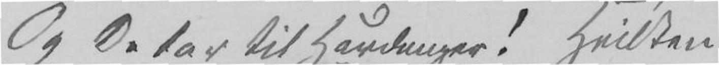Og De tar til Hardanger! Hvilken
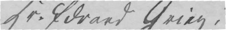Hr. Edvard Grieg!
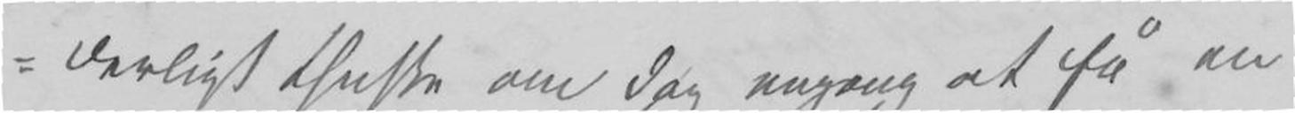=derligt Ønske om dog engang at få en
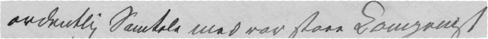ordentlig Samtale med vor store Komponist
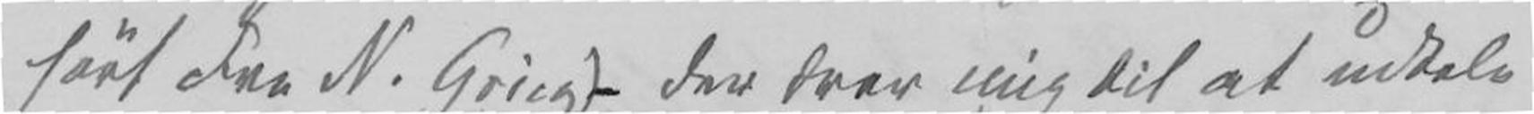hørt Fru N. Grieg) - der svar mig til at udtale
